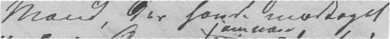Mænd, der havde modtaget
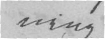ning
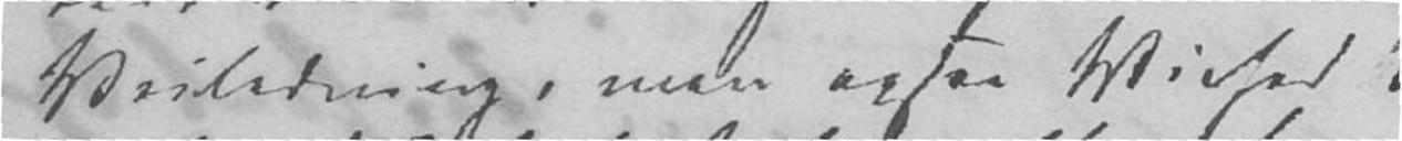Veiledning, men ogsaa Vished
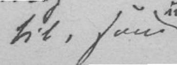til, som
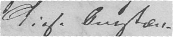Disse Omstæn-
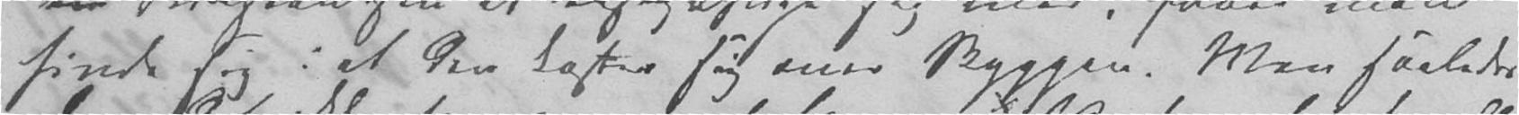finde sig i at den kaster sig over Skyggen. Men saaledes
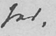hed,
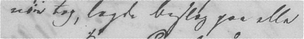veie tog, lagde Beslag paa alle
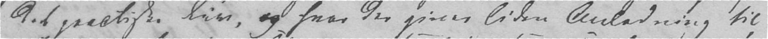det practiske Liv, og hvor der gives liden Anledning til
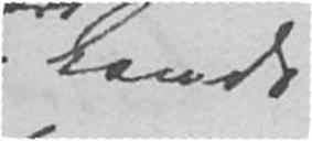Lands
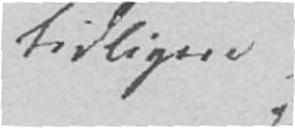tidligere
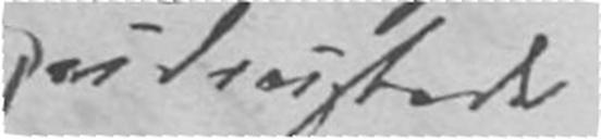udrustede
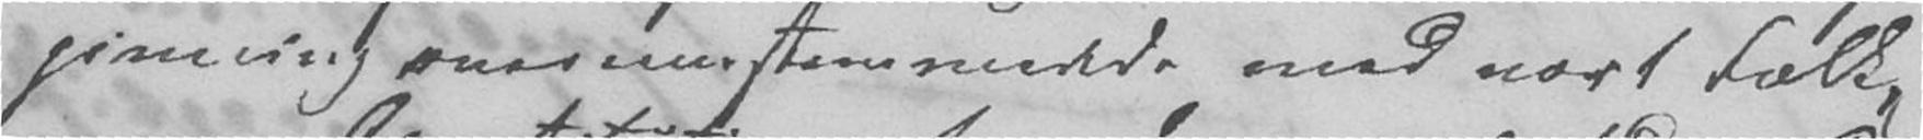givning overeenstemmende med vort Folk,
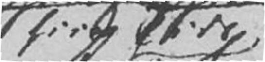i hiin Tids
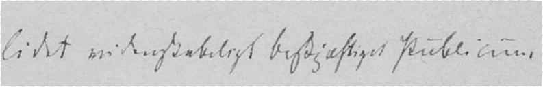lidet videnskabeligt beskjæftiget Publicum
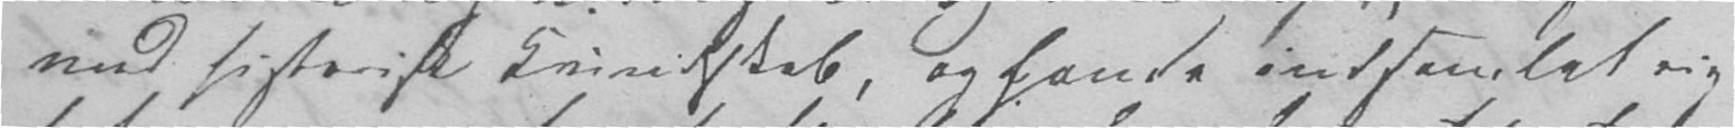med historisk Kundskab, og havde indsamlet rig
